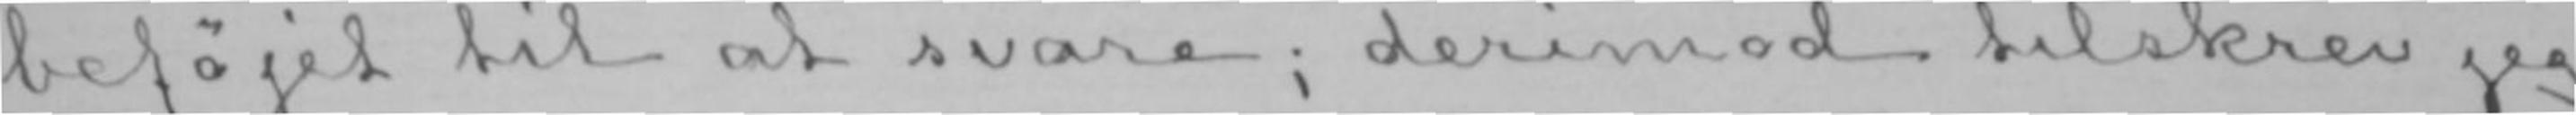beføjet til at svare; derimod tilskrev jeg
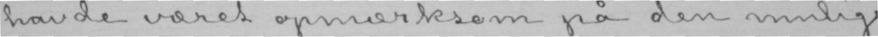havde været opmærksom på den mulige
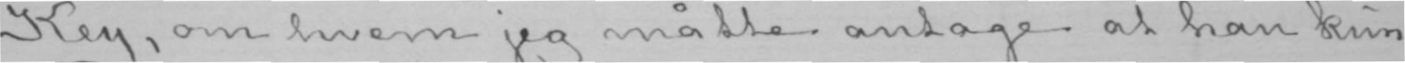Key, om hvem jeg måtte antage at han kun
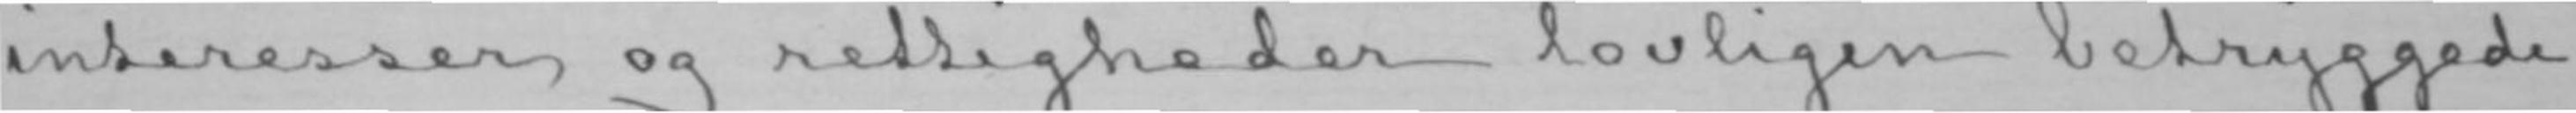interesser og rettigheder lovligen betryggede
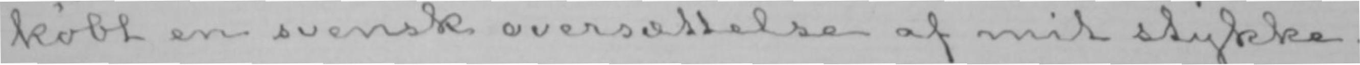købt en svensk oversættelse af mit stykke.
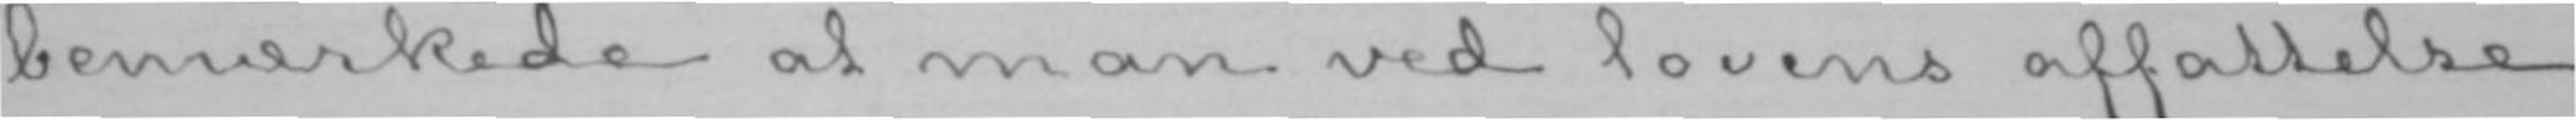bemærkede at man ved lovens affattelse
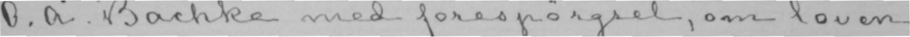O. A. Bachke med forespørgsel, om loven
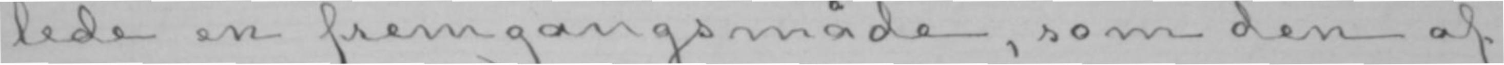lede en fremgangsmåde, som den af
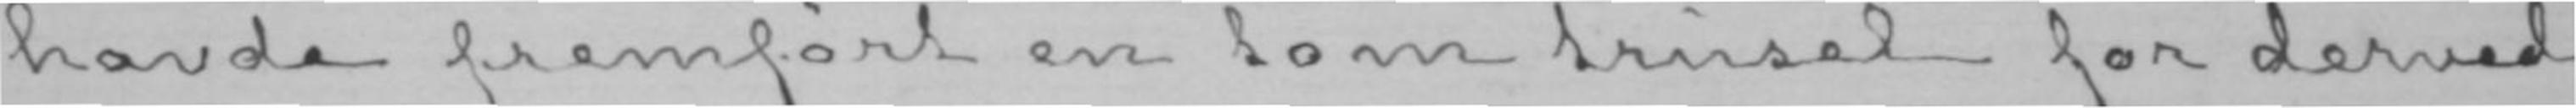havde fremført en tom trusel for derved
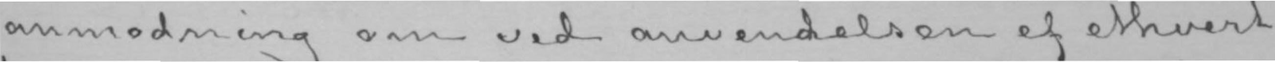anmodning om ved anvendelsen af ethvert
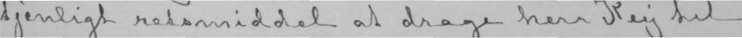tjenligt retsmiddel at drage herr Key til
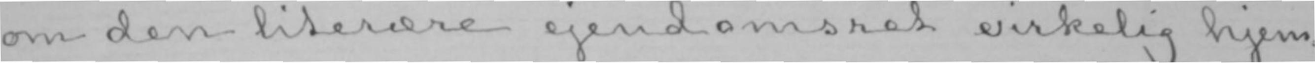om den literære ejendomsret virkelig hjem-
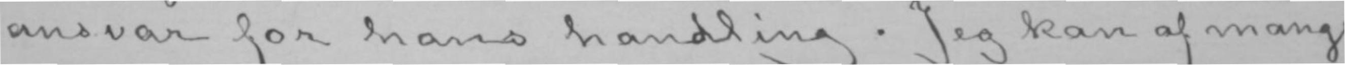ansvar for hans handling. Jeg kan af mange
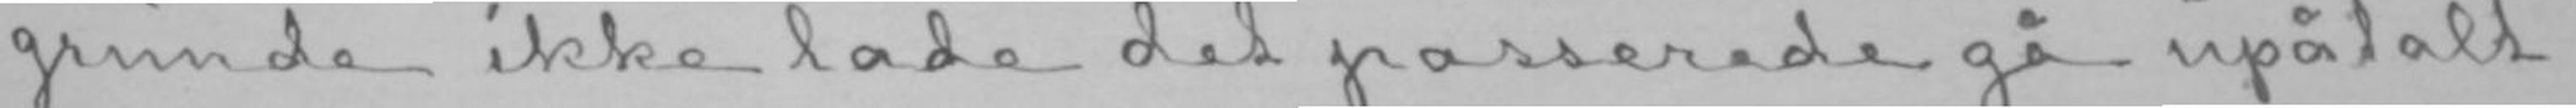grunde ikke lade det passerede gå upåtalt
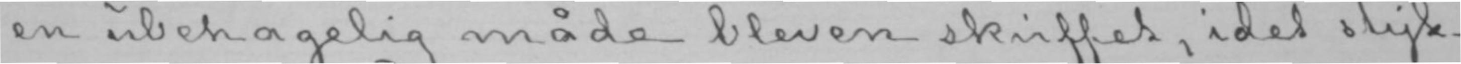en ubehagelig måde bleven skuffet, idet styk-
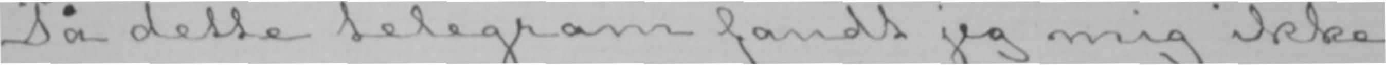På dette telegram fandt jeg mig ikke
